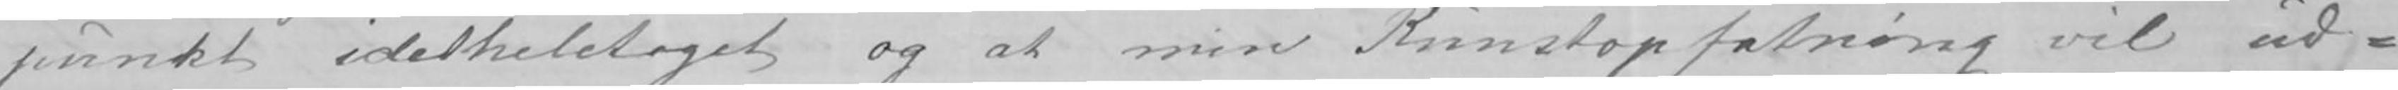punkt idetheletaget og at min Kunstopfatning vil ud-
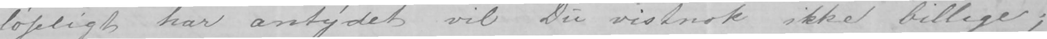løseligt har antydet vil Du vistnok ikke billige;
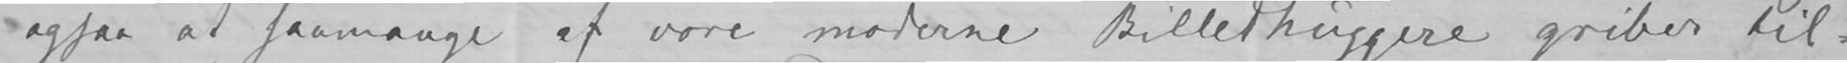ogsaa at saamange af vore moderne Billedhuggere griber til-
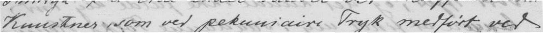Kunstner, som ved pekuniaire Tryk medført ved
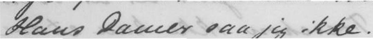Hans Damer saa jeg ikke.
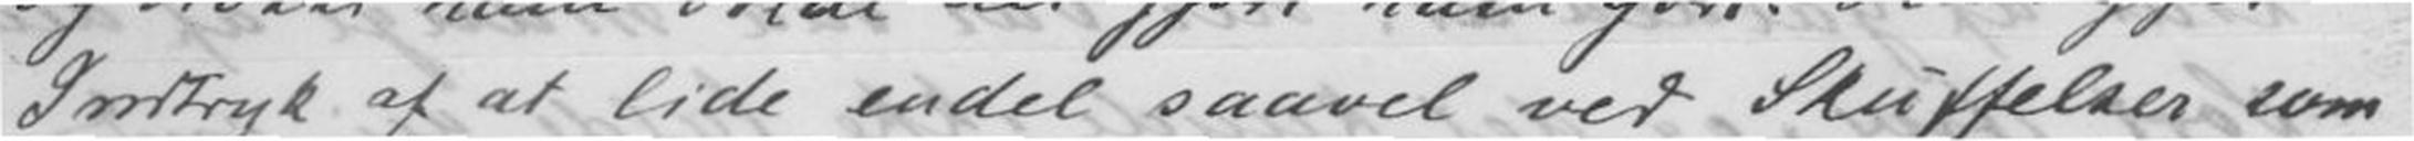Indtryk af at lide endel saavel ved Skuffelser som
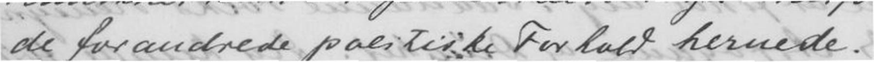de forandrere politiske Forhold hernede.
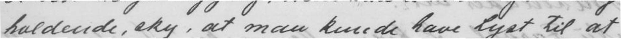holdende, sky, at man kunde have Lyst til at
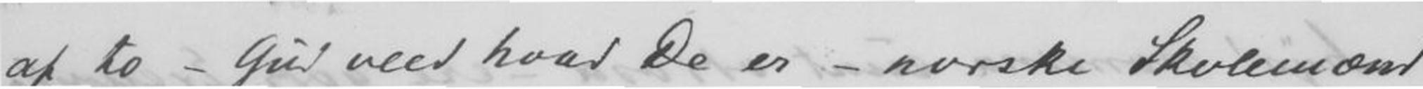af to - Gud veed hvad De er - norske Skolemænd
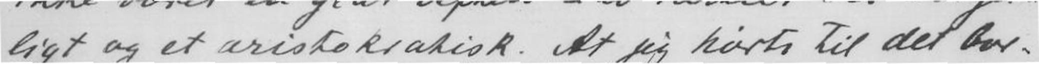ligt og et aristokratisk. At jeg hørte til det bor-
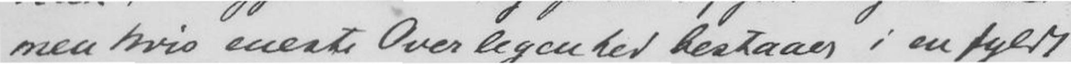men hvis eneste Overlegenhed bestaar i en fyldt
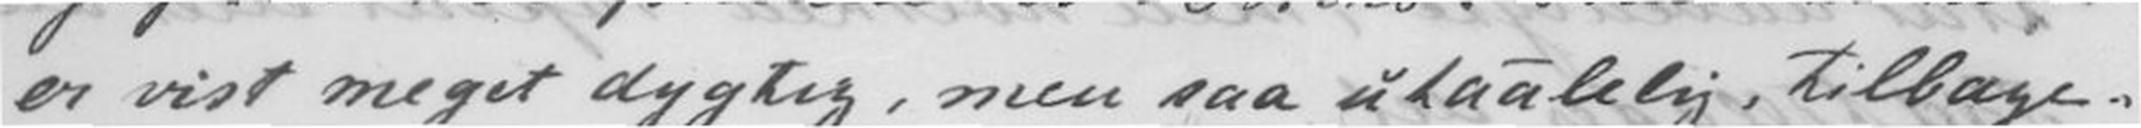er vist meget dygtig, men saa utaalelig tilbage-
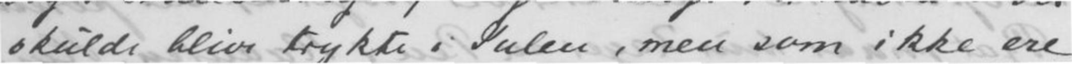skulde blive trykte i Julen, men som ikke ere
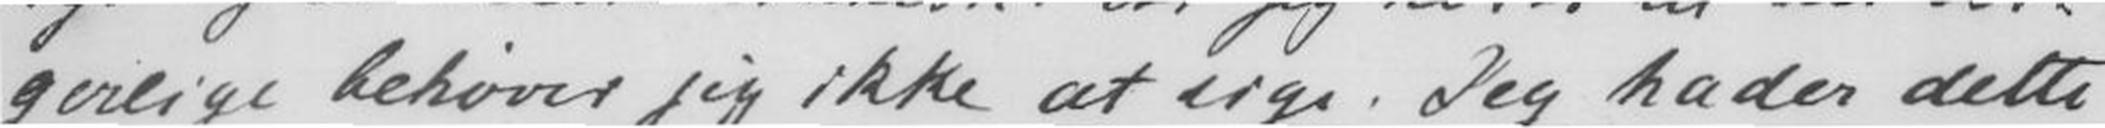gerlige behøver jeg ikke at sige. Jeg hader dette
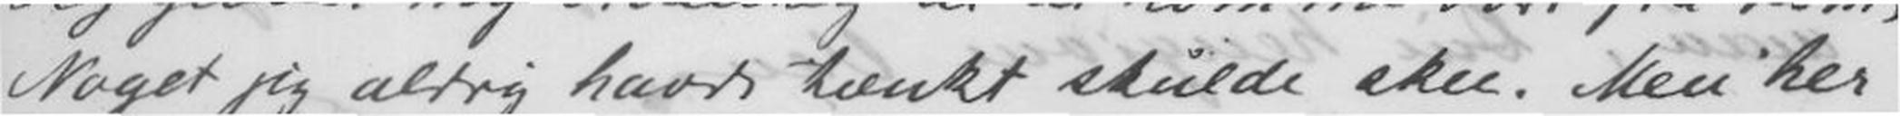Noget jeg aldrig havde tænkt skulde skee. Men her
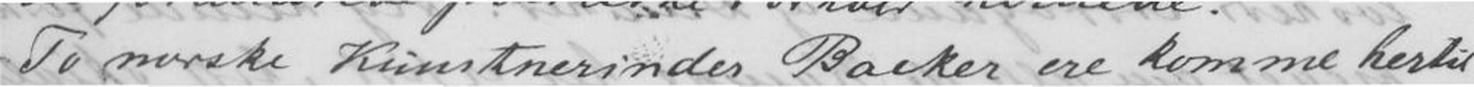To norske Kunstnerinder Backer ere komme hertil
